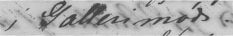i Gallerimøde.
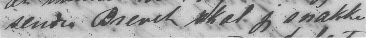sender Brevet skal jeg snakke
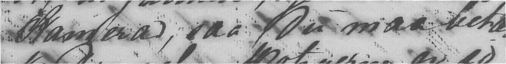Kamerad, saa Du maa betæn-
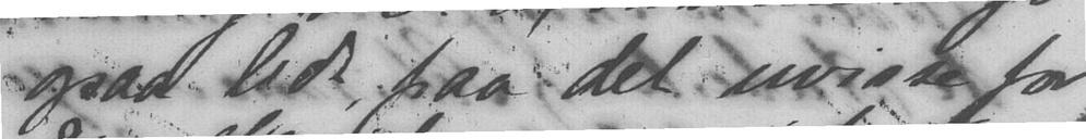ogsaa lidt paa det uvisse for
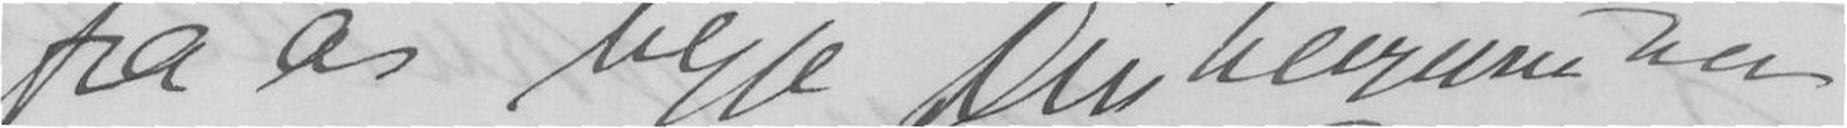Din hengivne ven fra os begge.
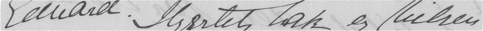Edvard. Hjærtelig tak og hilsen
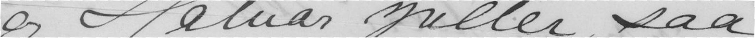og Halvar spiller, saa
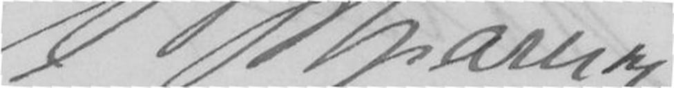B Bjørnson
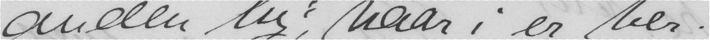andenlig, Naar i er her.
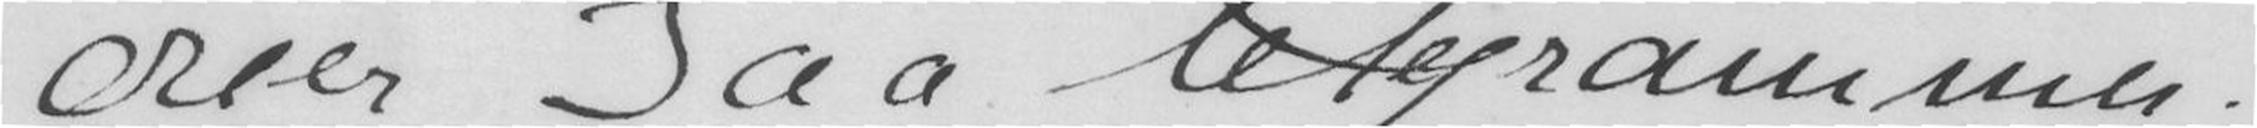over Ja a telegrammer.
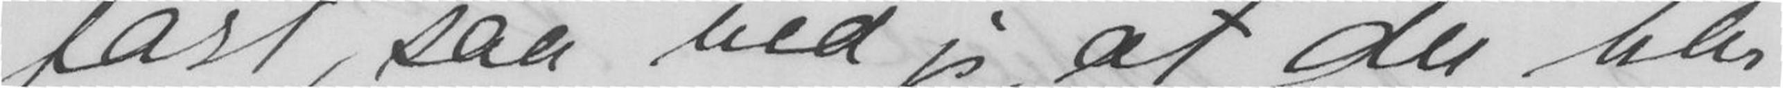fast, saa ved jeg, at du blir
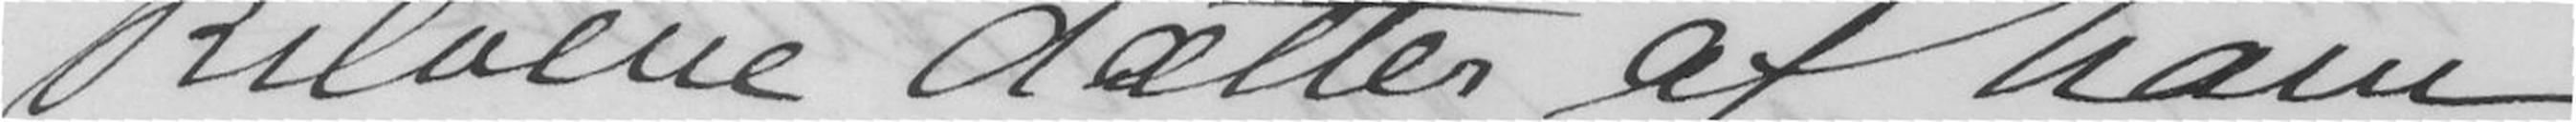kiloene dætter af ham
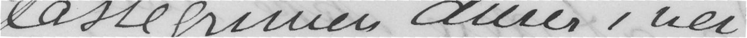- Fossegrimen durer i vei
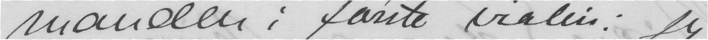manden i første violin: jeg
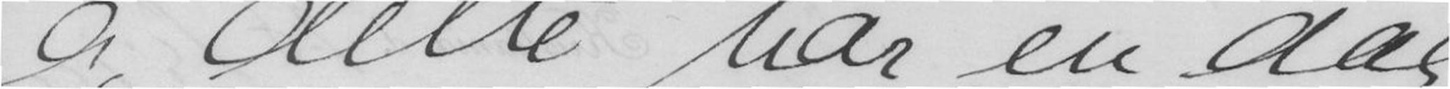Ja, dette har en dag
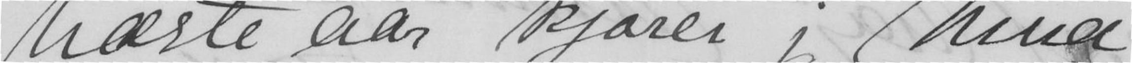Næste aar kjorer jeg Nina
